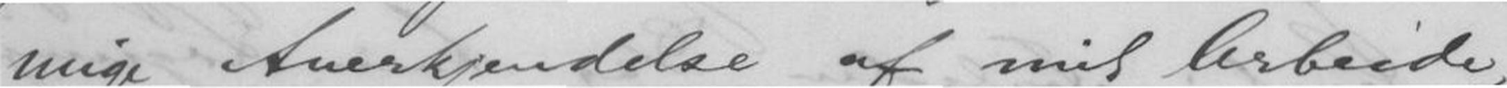mige Anerkjendelse af mit Arbeide,
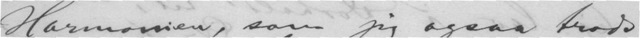Harmonien, som jeg ogsaa trods
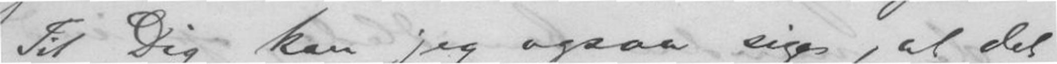Til Dig kan jeg ogsaa sige, at det
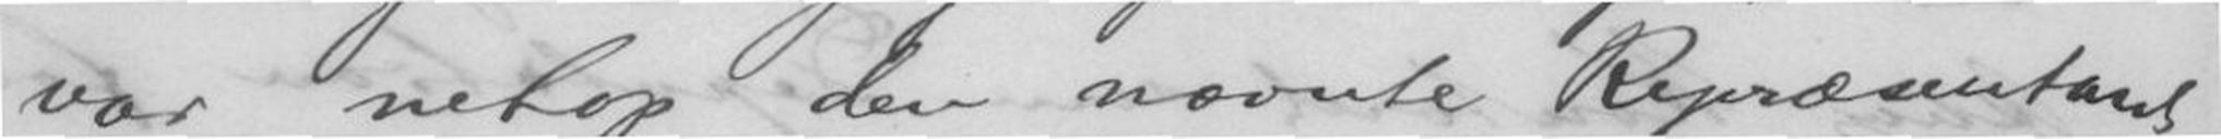var netop den nævnte Repræsentant
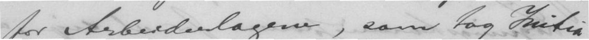for Arbeiderlagene, som tog Initia-
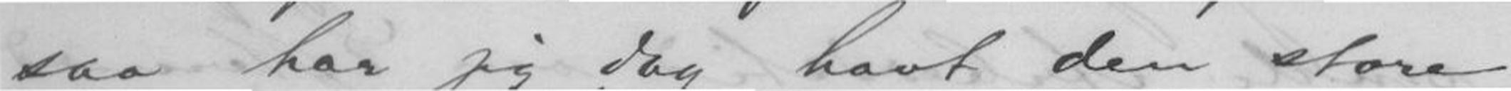saa har jeg dog havt den store
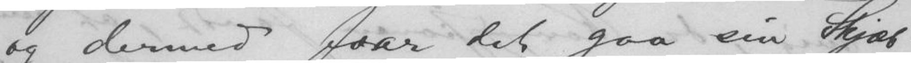og dermed faar det gaa sin Skjæb-
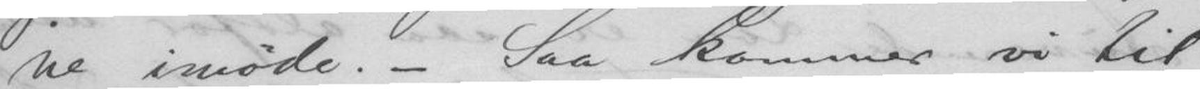ne imøde. - Saa kommer vi til
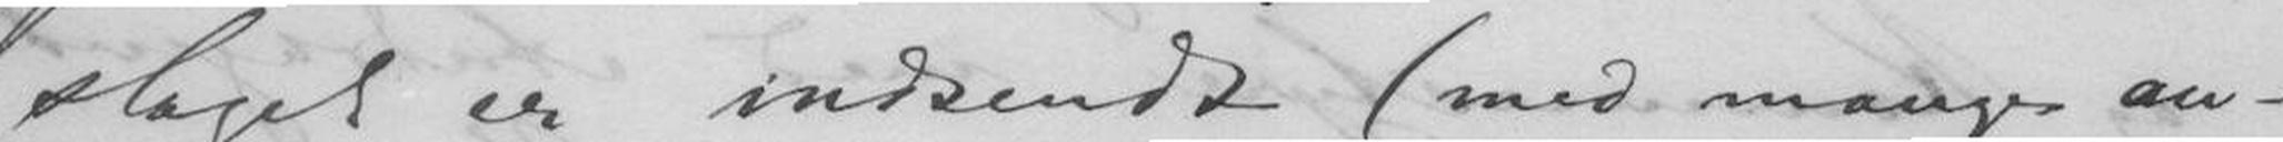slaget er indsendt (med mange an-
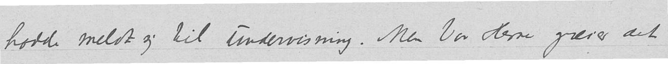hadde meldt sig til undervisning. Men Vor Herre greier det
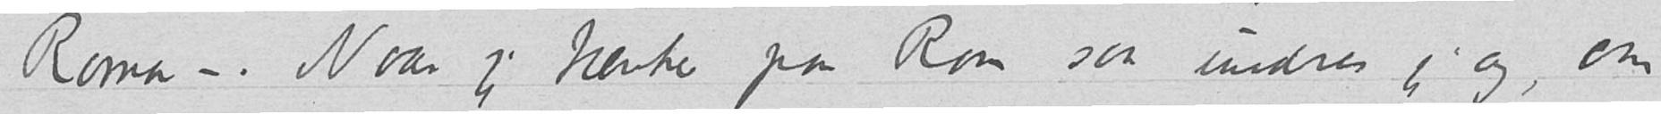Roma –. Naar jeg tænker paa Rom saa undres jeg og, om
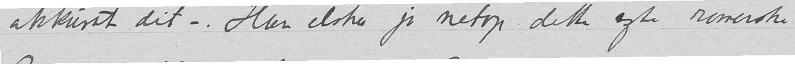akkurat dit –. Han elsker jo netop dette ægte romerske
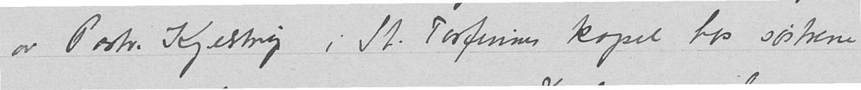av Pastor Kjelstrup i St. Torfinns kapel hos søstrene
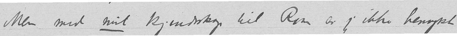Men med mit kjendskap til Rom er jeg ikke henrykt
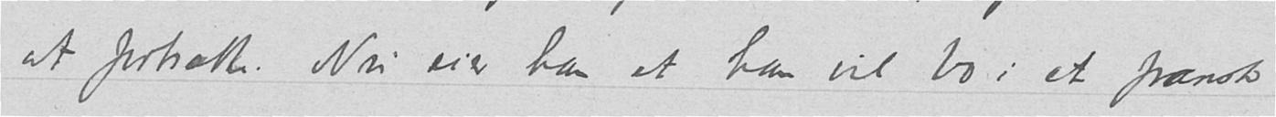at fortsætte. Nu sier han at han vil bo i et fransk
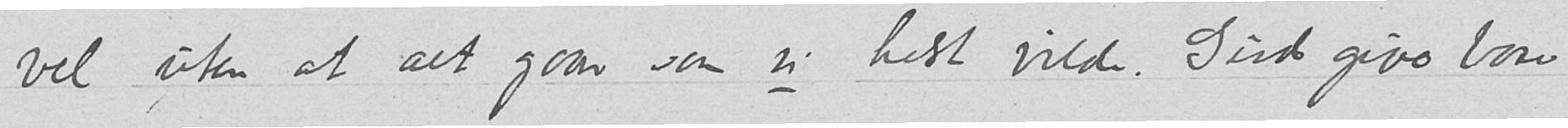vel uten at alt gaar som vi helst vilde. Gud give bare
